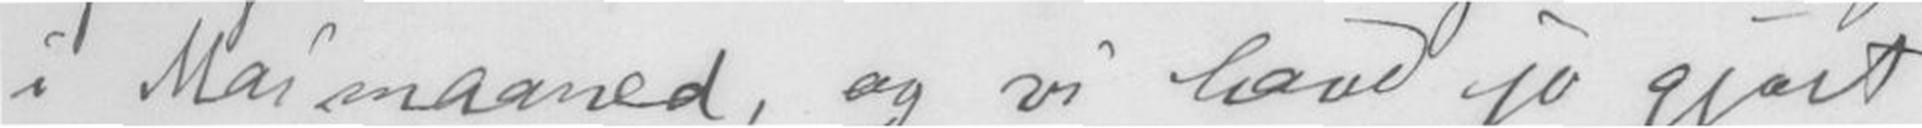i Mai maaned, og vi have jo gjort
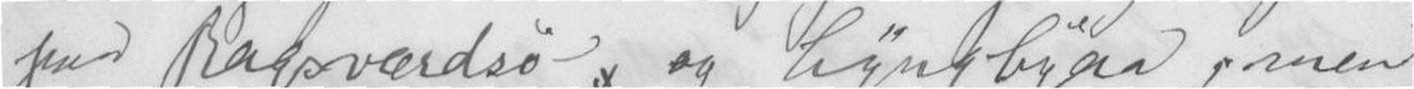paa Bagsværdsø - og Lyngbyaa, men
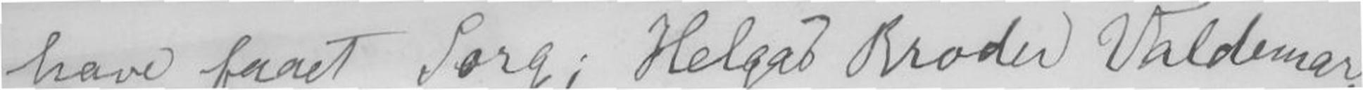have faaet Sorg; Helgas Broder Valdemar,
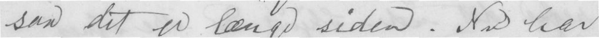saa det er længe siden. Nu har
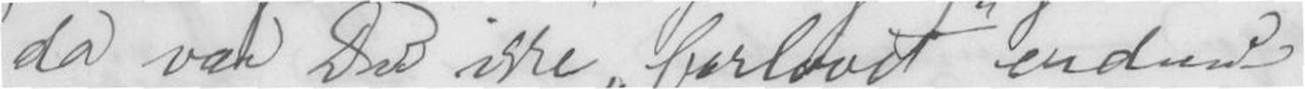da var Du ikke forlovet endnu,
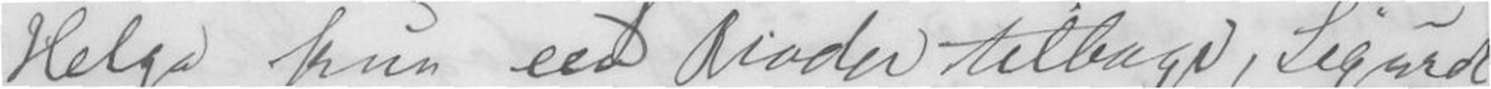Helga kun en Broder tilbage, Sigurd,
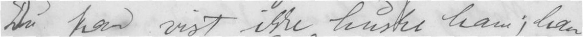Du kan vist ikke huske ham; han
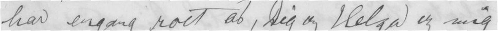har engang roet os, Dig og Helga og mig
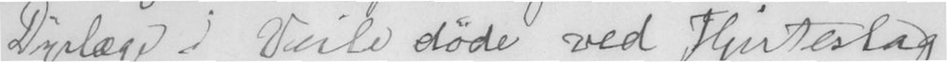Dyrlæge i Veile døde ved Hjerteslag
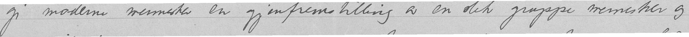gi moderne mennesker en gjenfremstilling av en slik gruppe mennesker og
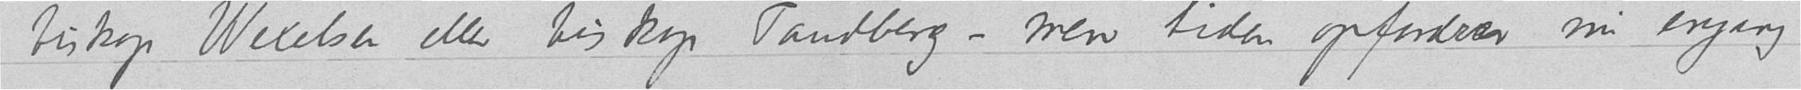biskop Wexelsen eller biskop Tandberg – men tiden opforder nu engang
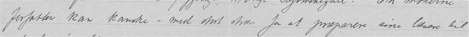forfatter kan kanske – med stort stræv for at præparere sine læsere til
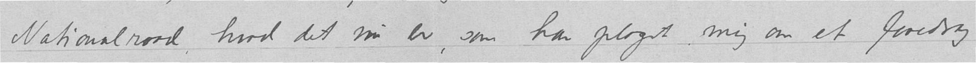Nationalraad, hvad det nu er, som har plaget mig om et foredrag
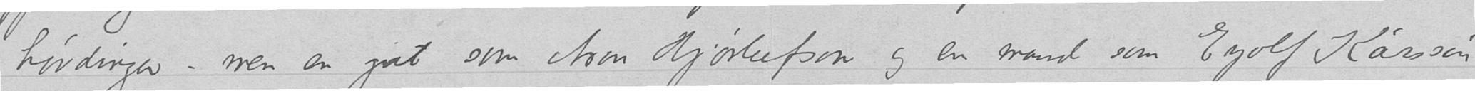høvdinger – men en gut som Aron Hjorlufson og en mand som Eyolf Karsson
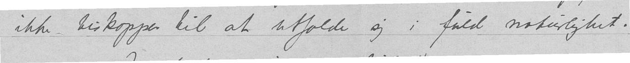ikke biskopper til at utfolde sig i fuld naturlighet.
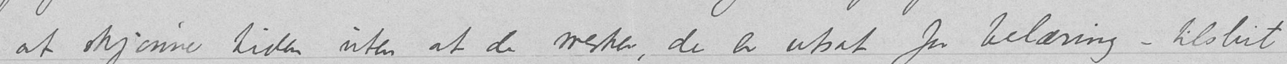at skjønne tiden uten at de merker, de er utsat for belæring – tilslut
